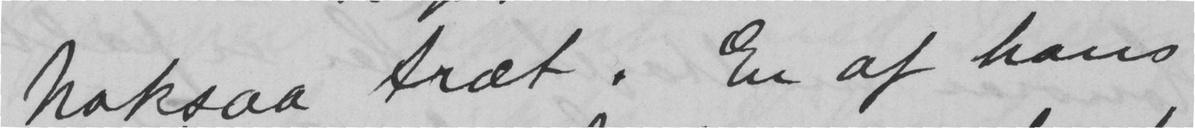Noksaa træt. En af hans
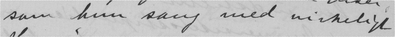som hun sang med virkeligt
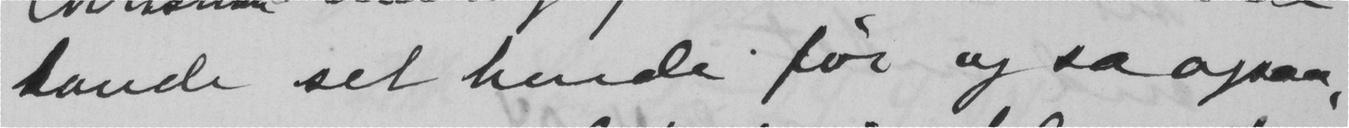havde set hende før og sa ogsaa,
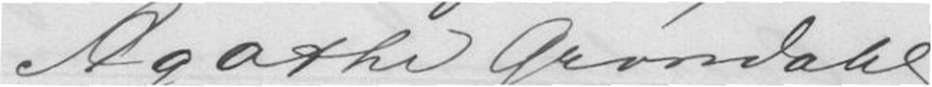Agathe Grøndahl
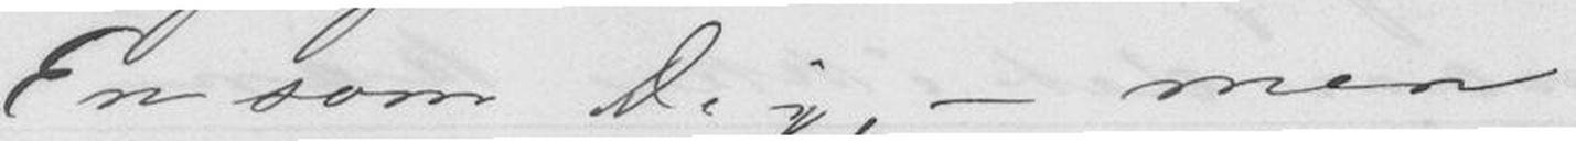En som Dig,- men
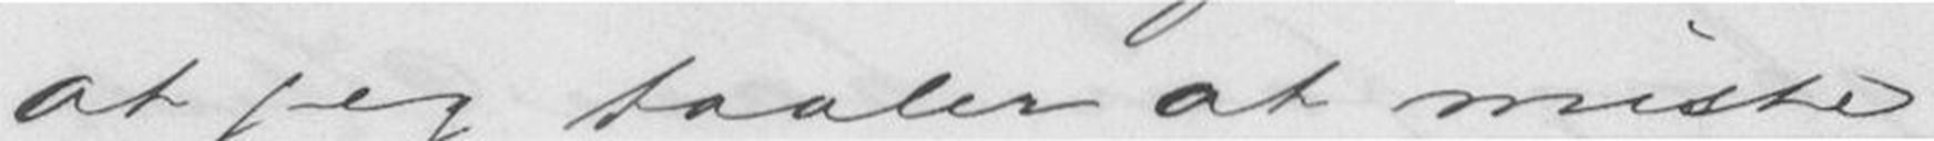at jeg taaler at miste
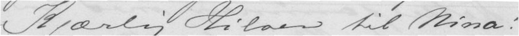Kjærlig Hilsen til Nina!
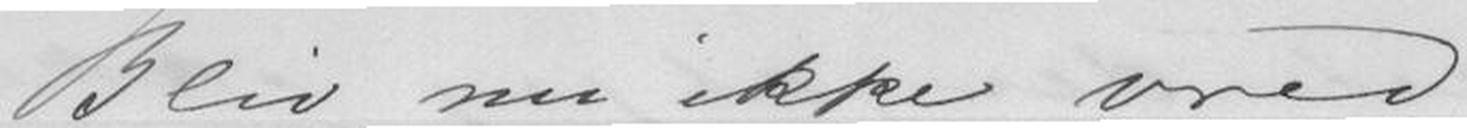Bli nu ikke vred
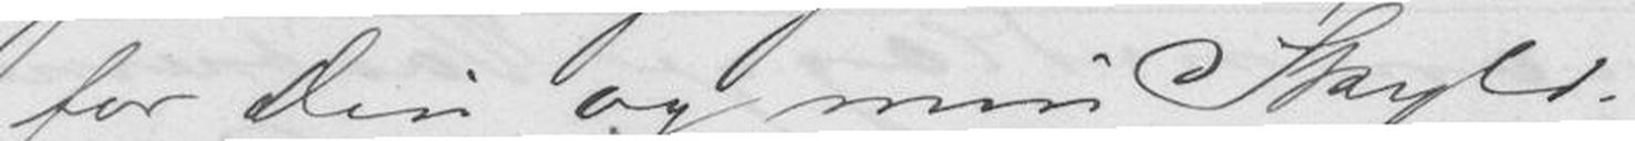for Din og min Skyld.
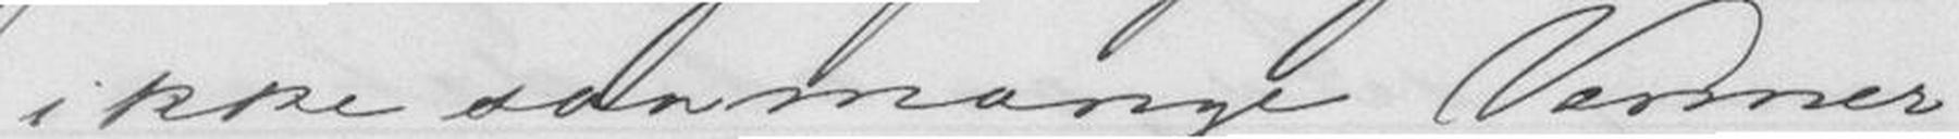ikke saamange Venner
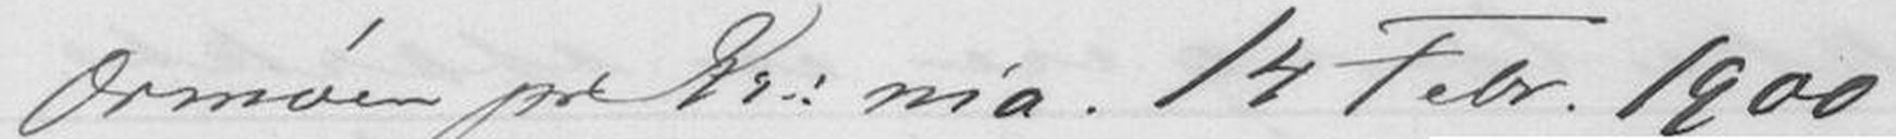Ormøen pr Kr.:nia. 14 Febr. 1900
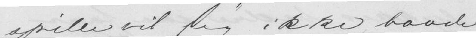spille vil jeg ikke baade
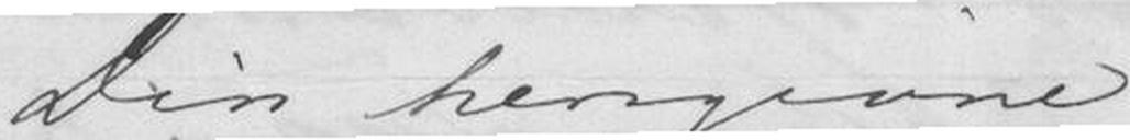Din hengivne
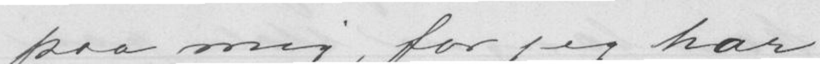paa mig, for jeg har
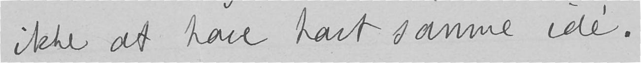ikke at have havt samme idé.
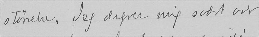størrelse. Jeg ærgrer mig svært over
